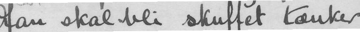Han skal bli skuffet tænker
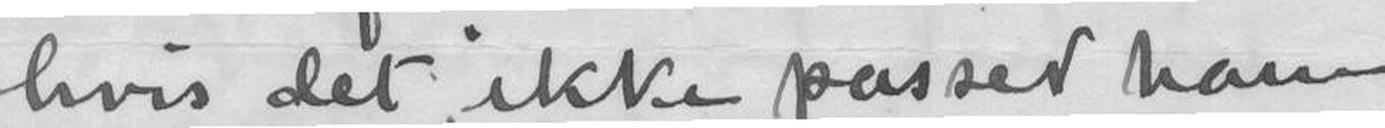hvis det ikke passer han
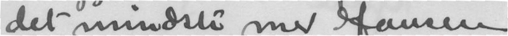det mindste med Hansen
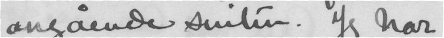angående suiten. Jeg har
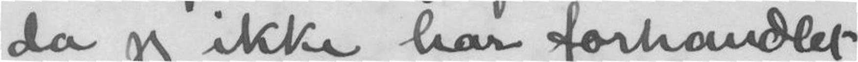da jeg ikke har forhandlet
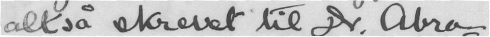altså skrevet til Dr. Abra-
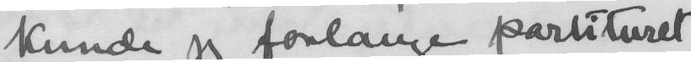kunde jeg forlange partituret
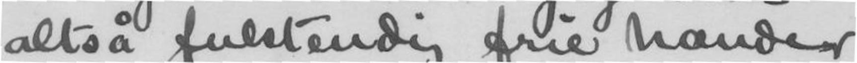altså fulstendig frie hænder
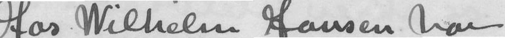Hos Wilhelm Hansen har
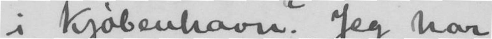i Kjøbenhavn? Jeg har
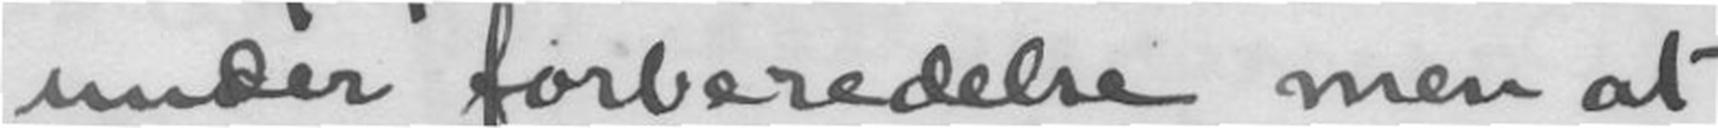under forberedelse men at
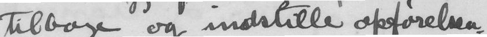tilbage og indstille opførelsen.
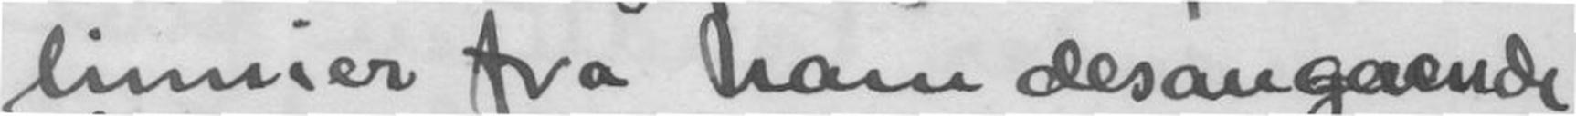linnier fra ham desangående
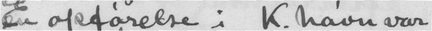En opførelse i K.havn var
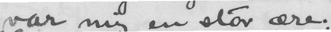var mig en stor ære;
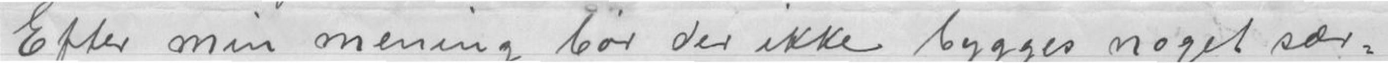Efter min mening bør der ikke bygges noget sær-
